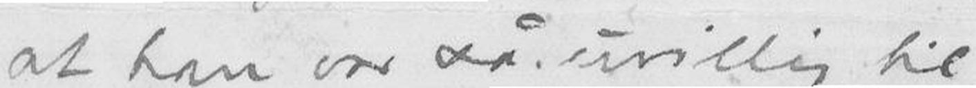at han var så uvillig til
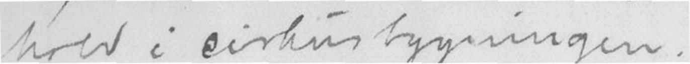hold i cirkus bygningen.
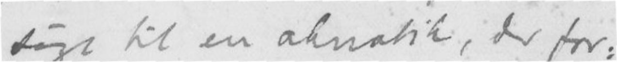sige til en akustik, du for-
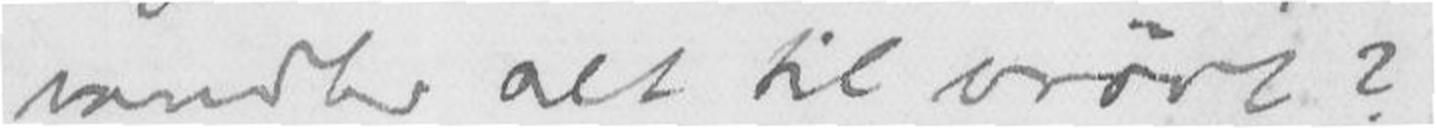vandler alt til vrøvl?
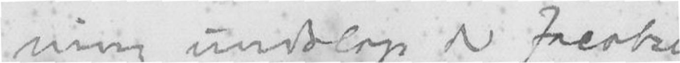ning undslap du Jacobsen,
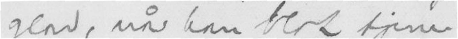glad, når han blot tjener
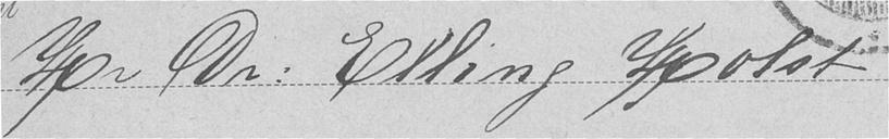Hr Dr. Elling Holst
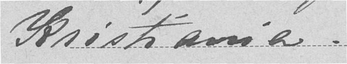Kristiania.
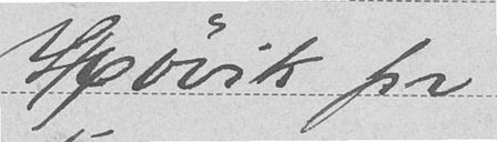Høvik pr
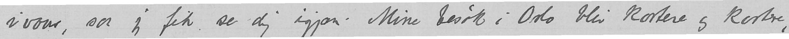ivaar, saa jeg fik se dig igjen. Mine besøk i Oslo blir kortere og kortere,
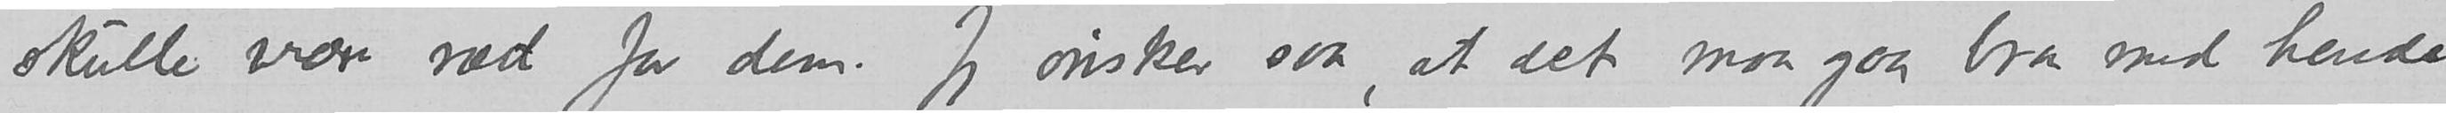skulle være ræd for dem. Jeg ønsker saa, at det maa gaa bra med hende
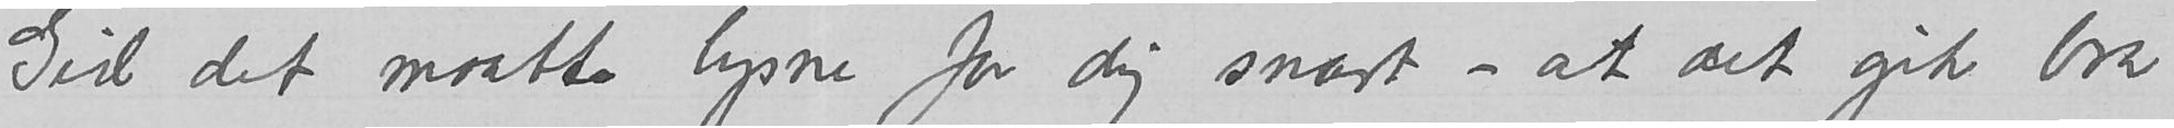Gid det maate lysne for dig snart – at det gik bra
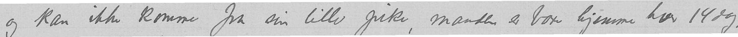og kan ikke komme fra sin lille pike, manden er bare hjemme hver 14 dag,
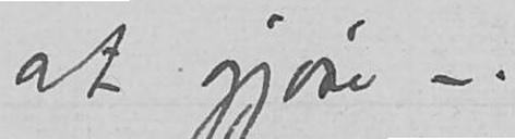at gjøre –.
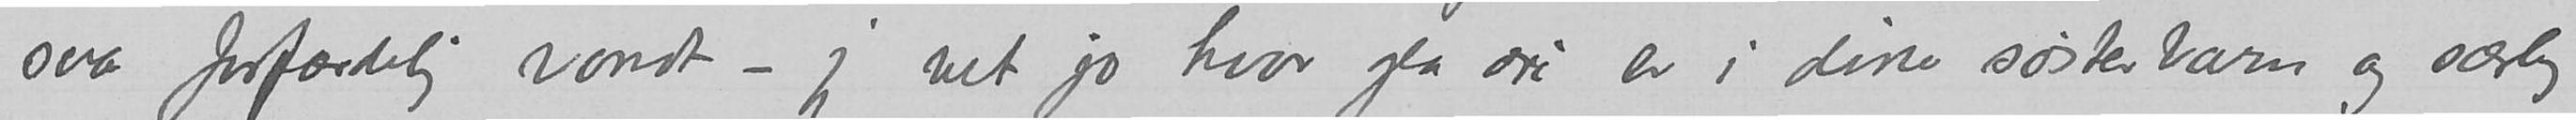saa forfærdelig vondt – jeg vet jo hvor gla du er i dine søsterbarn og særlig
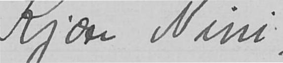Kjære Nini,
